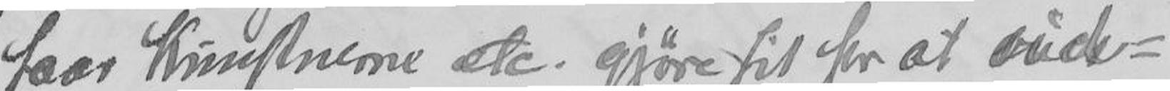faar Kunstnerne etc. gjøre sit for at bud-
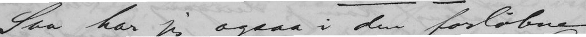Saa har jeg ogsaa i den forløbne
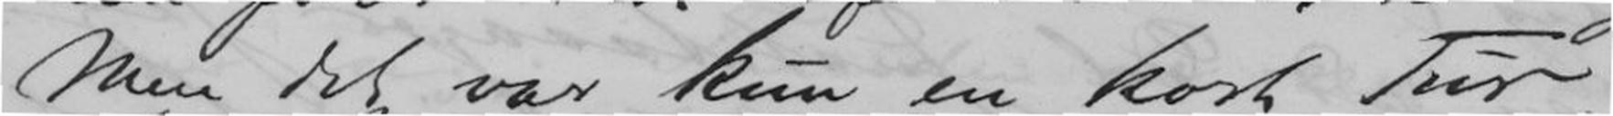Men det var kun en kort Tur, -
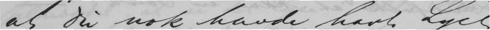at Du nok havde havt Lyst
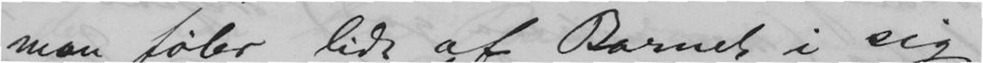man føler lidt af Barnet i sig.
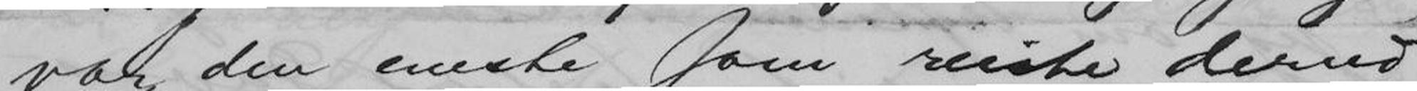var den eneste som reiste derud
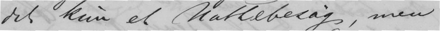det kun et Nattebesøg, men
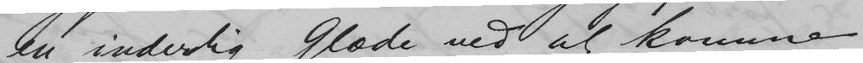en inderlig Glæde ved at komme
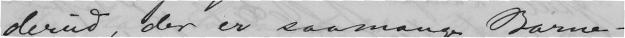derud, der er saamange Barne-
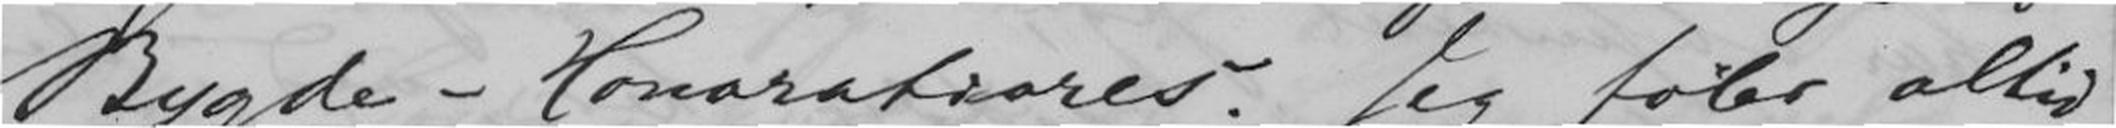Bygde-Honoratiores. Jeg føler altid
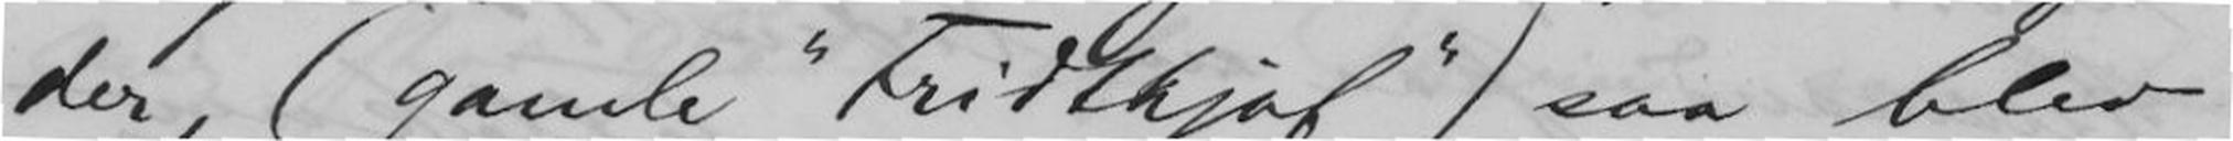der, (gamle "Fridthjof") saa blev
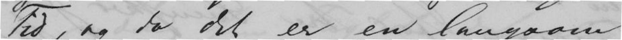Tid, og da det er langsom
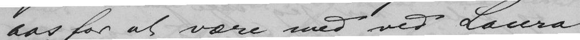aas for at være med ved Laura
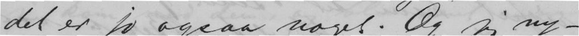det er jo ogsaa noget. Og jeg ny-
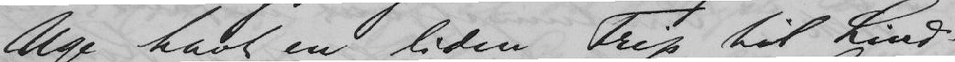Uge havt en liden Trip til Lind-
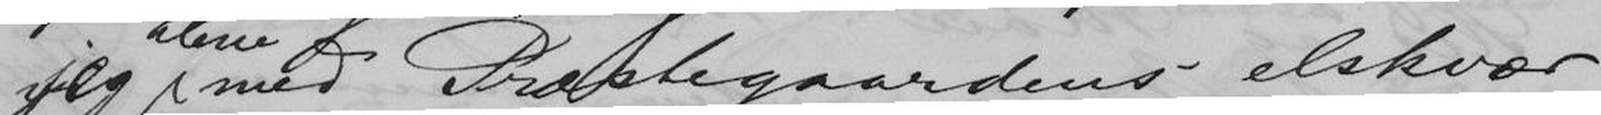jeg med Præstegaardens elskvær-
